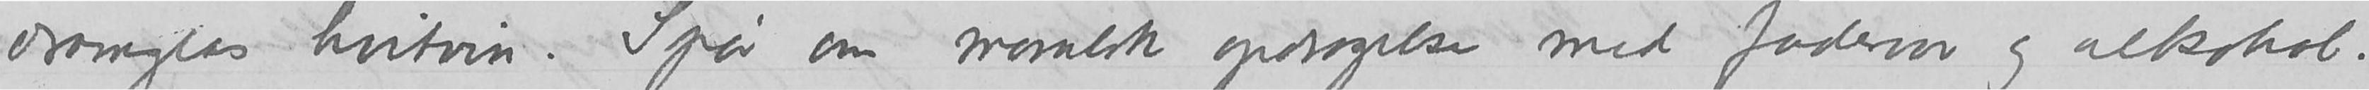dramglas hvitvin. Spør om moralsk opdragelse med fadervaar og alkohol.
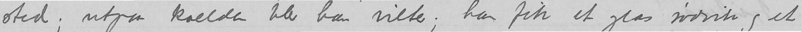sted; utpaa kvelden blev han vilter; han fik et glas rødvin og et
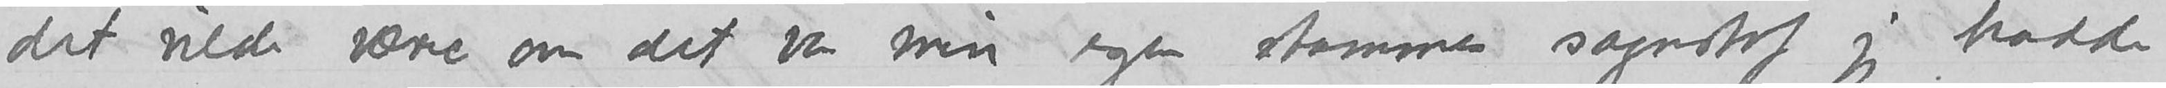det vilde være om det var min egen stammes sagastof jeg hadde
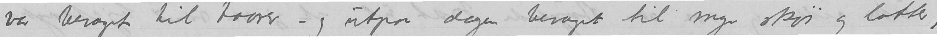var bevæget til taarer – og utpaa dagen bevæget til mye skøi og latter;
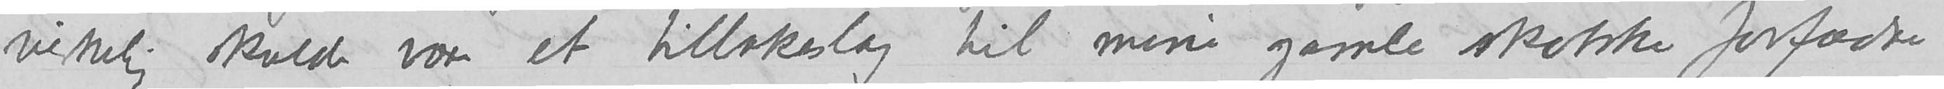virkelig skulde være et tilbakeslag til mine gamle skotske forfædre –
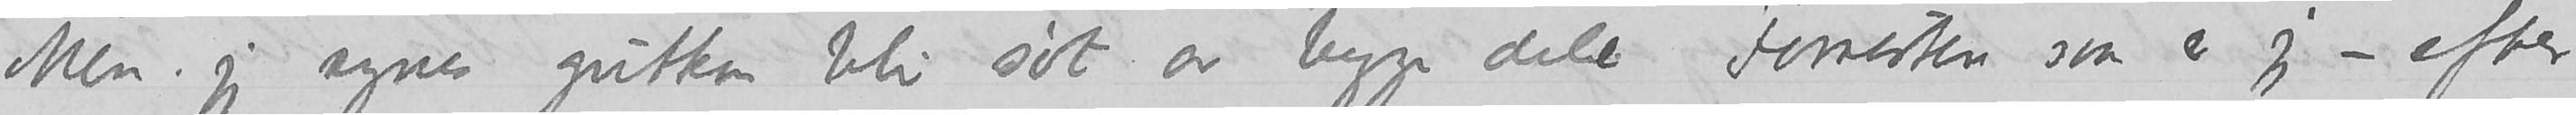Men jeg synes gutten blir søt av begge dele. Forresten saa er jeg – efter
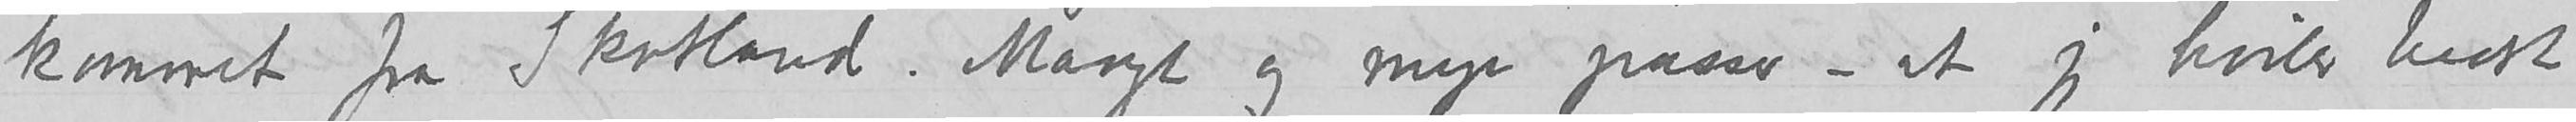kommet fra Skotland. Mangt og mye passer – at jeg hviler bedst
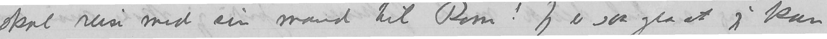skal reise med sin mand til Rom! Jeg er saa gla at jeg kan
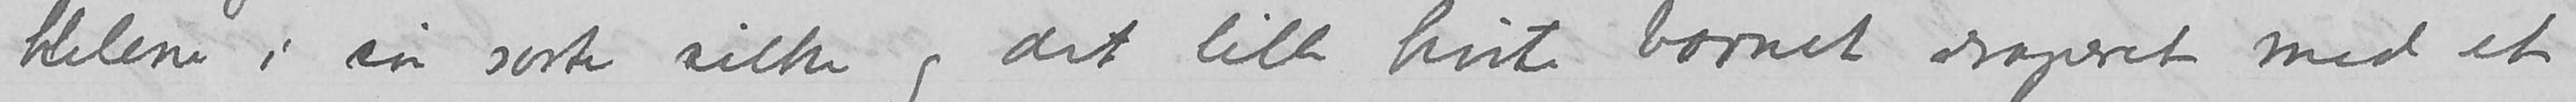Helene i sin sorte silke og det lille hvite barnet draperet med et
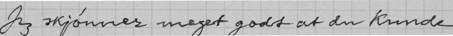Jeg skjønner meget godt at du kunde
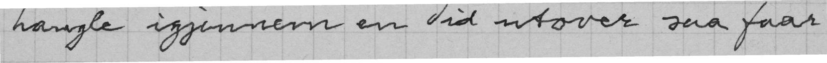hangle igjennem en Tid utover saa faar
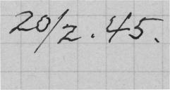20/2. 45.
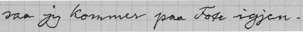saa jeg kommer paa Fote igjen.
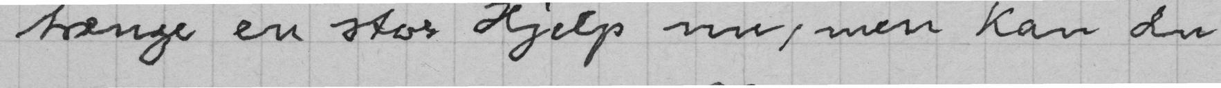trænge en stor Hjelp nu, men kan du
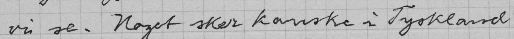vi se. Noget sker kanske i Tyskland
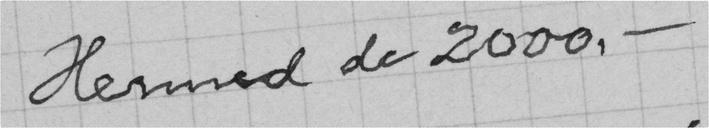Hermed de 2000. -
